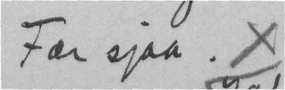Fær sjaa. x
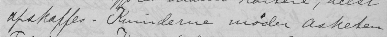afskaffes. Kvinderne møder Asketen
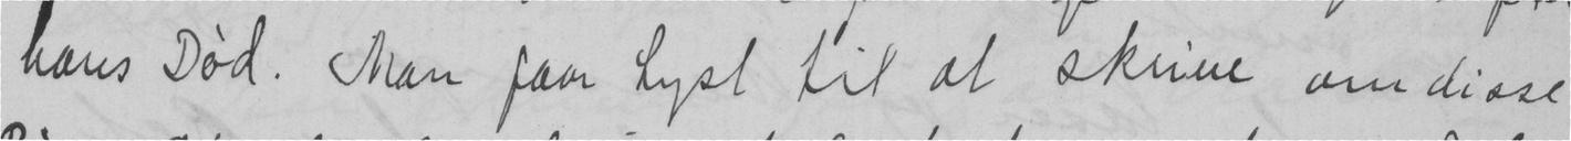hans Død. Man faar Lyst til at skrive om disse
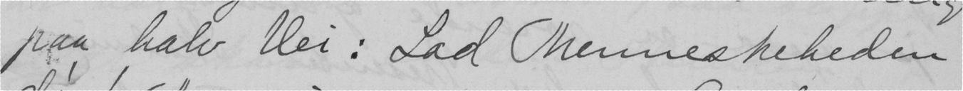paa halv vei: Lad menneskeheden
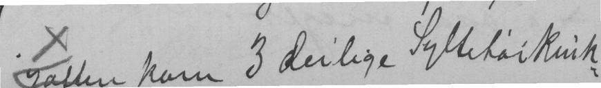I aften kom 3 deilige Syltetørkruk-
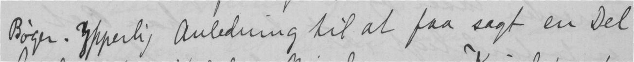Bøger. Ypperlig Anledning til at faa sagt en Del
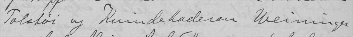Tolstoi og Kvindehaderen Weininger
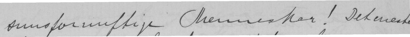snusfornftige mennesker! Det eneste
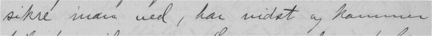sikre man ved, har vidst og kommer
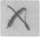x
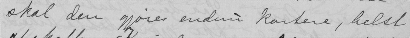skal den gjøres endnu kortere, helst
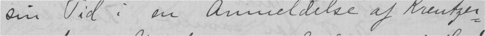sin Tid i en anmeldelse af Kreutzer-
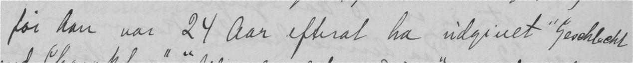før han var 24 Aar efterat ha udgivet "Geschlecht
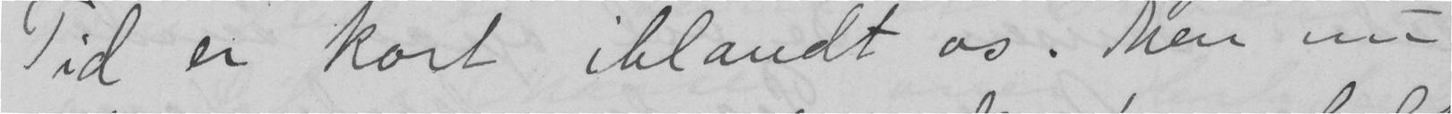Tid er kort iblandt os. Men nu
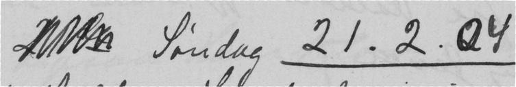Søndag 21.2.04
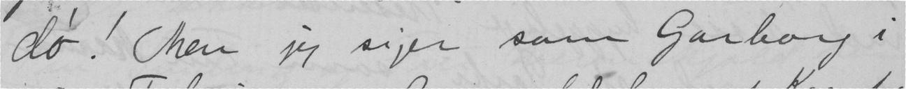dø! Men jeg siger som Garborg i
